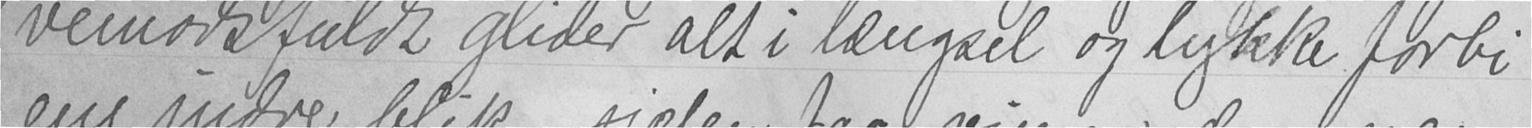vemodsfuldt glider alt i længsel og lykke forbi
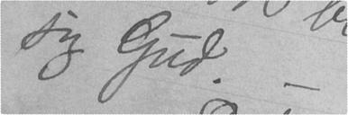sig Gud. -
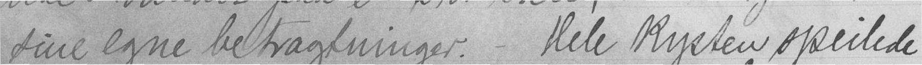sine egne betragtninger. - Hele kysten speilede
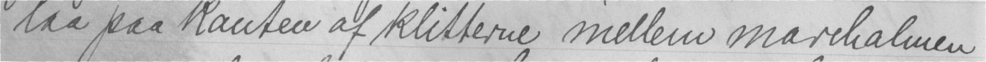laa paa kanten af klitterne mellem marehalmen
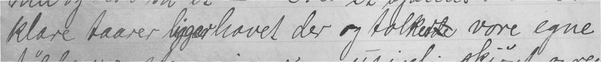klare taarer ligger havet der og tolkede vore egne
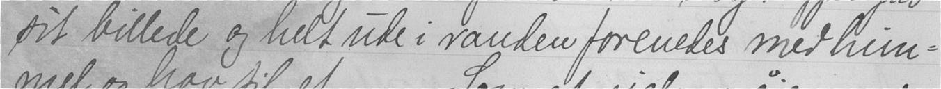sit billede og helt ude i randen forenedes med him-
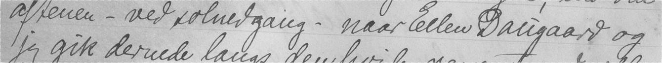aftenen - ved solnedgang - naar Ellen Daugaard og
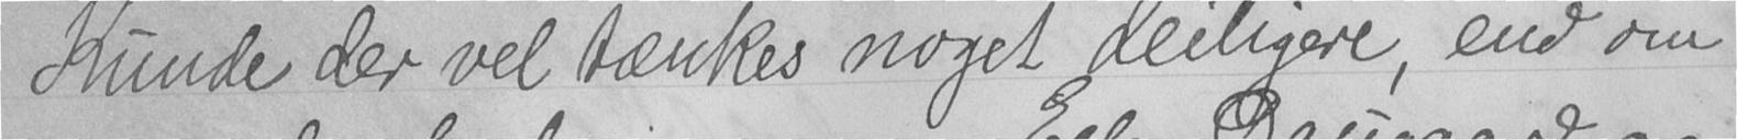Kunde der vel tænkes noget deiligere, end om
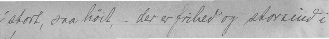stort, saa høit, - der er frihed og storsind i
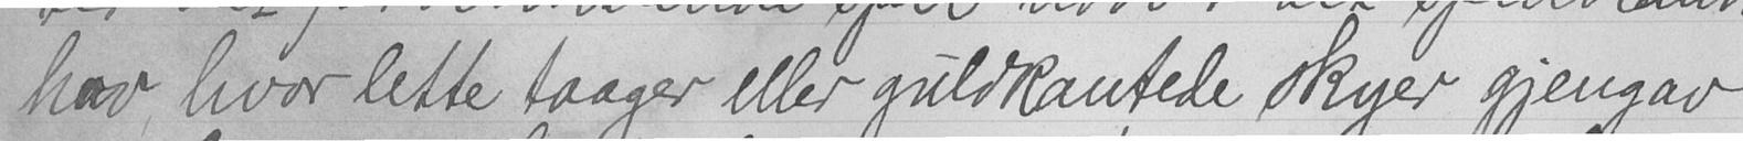hav, hvor lette taager eller guldkantede skyer gjengav
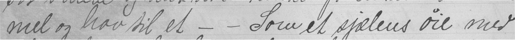mel og hav til et - - Som et sjælens øie med
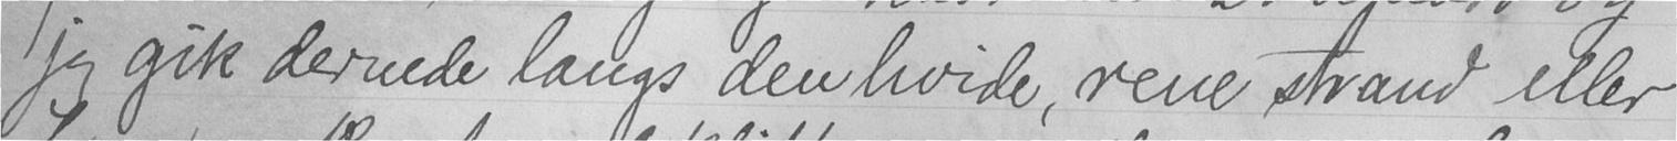jeg gik dernede langs den hvide, rene strand eller
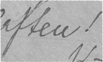luften!
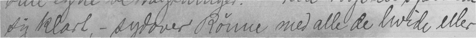sig klart, - sydover Rønne med alle de hvide eller
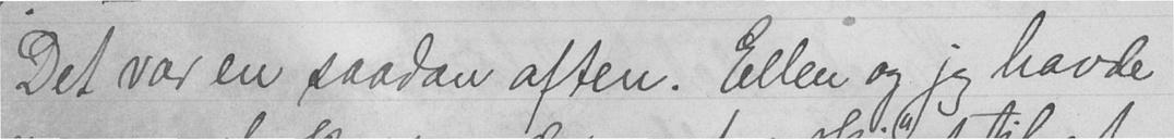Det var en saadan aften. Ellen og jeg havde
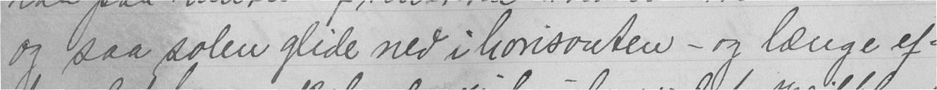og saa solen glide ned i horisonten - og længe ef-
